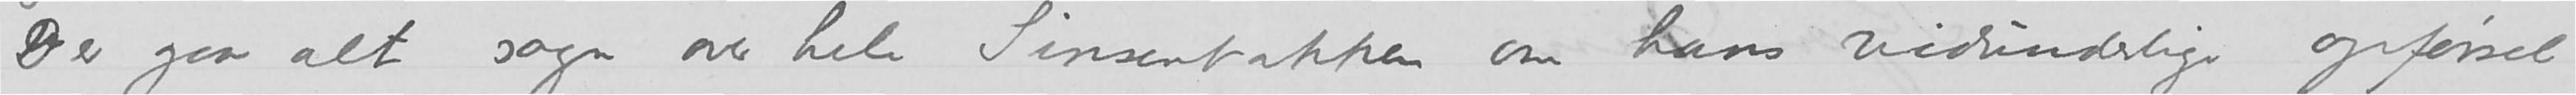Der gaar alt sagn over hele Sinsenbakken om hans vidunderlige opførsel
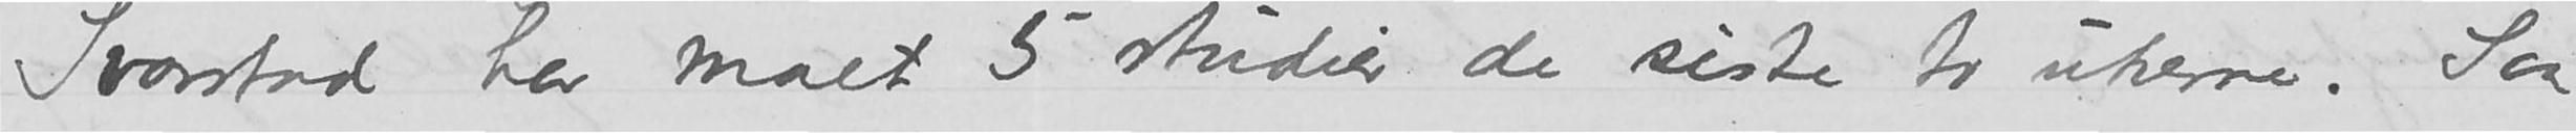Svarstad har malt 5 studier de siste to ukerne. Saa
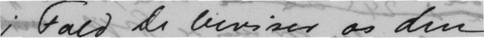i Fald De beviser os den
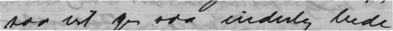saa vil jeg saa inderlig bede
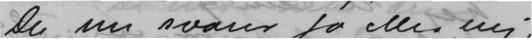Du nu svarer ja eller nej,
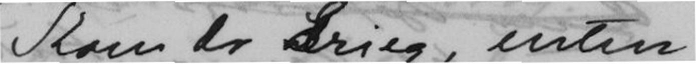Kære dr Grieg, enten
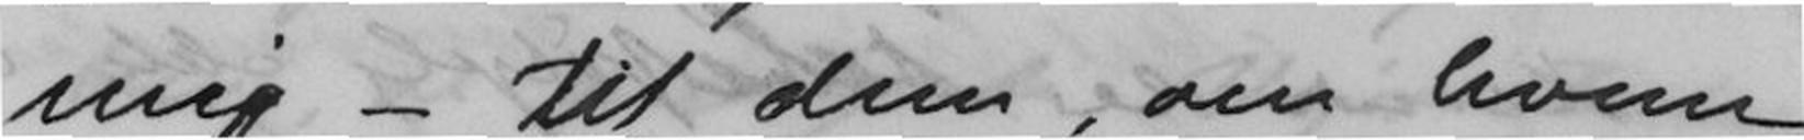mig - til dem, om hvem
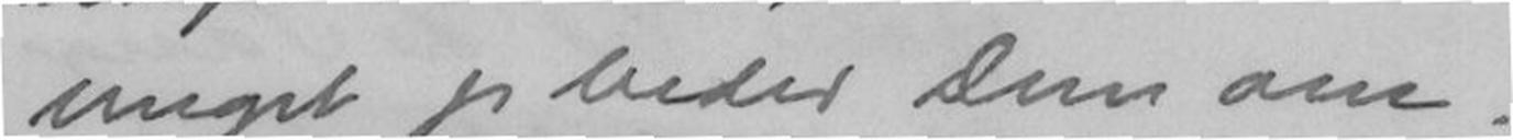meget jeg beder Dem om.
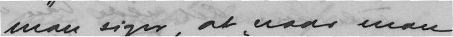man siger, at naar man
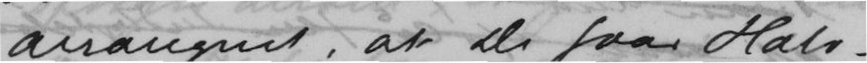arrangeret, at De faar Halv-
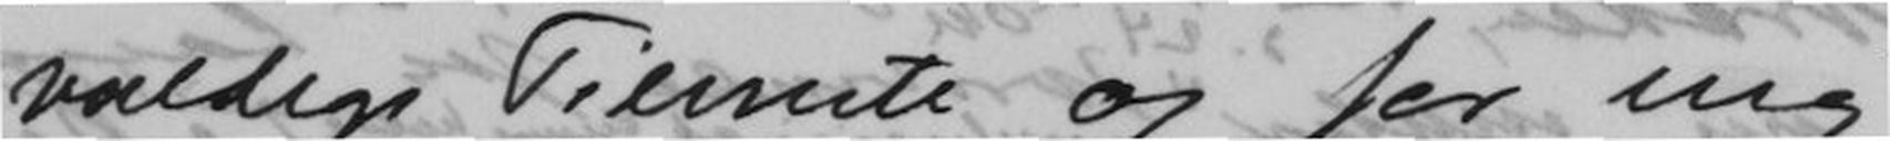vældige Tieneste og for mig
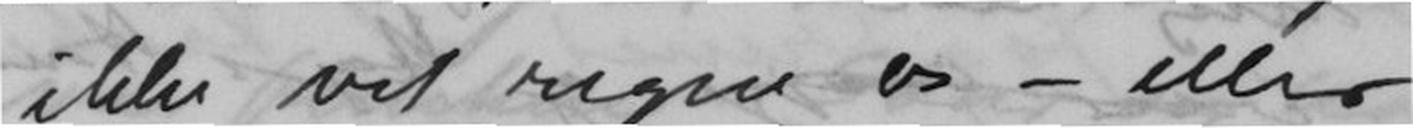ikke vil regne os - eller
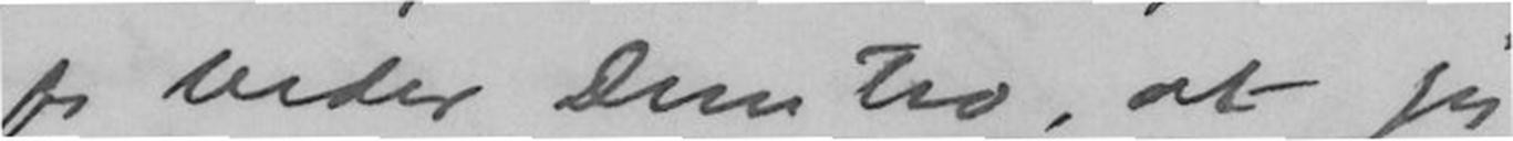jeg beder Dem tro, at jeg
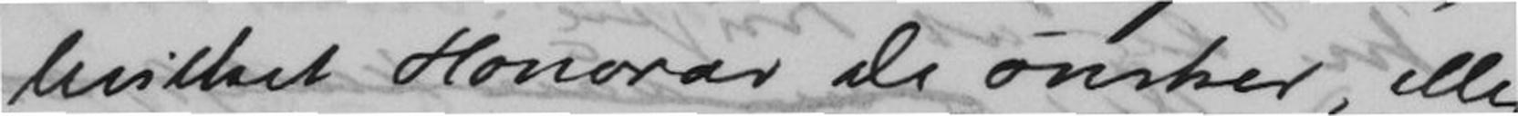hvilket Honorar De ønsker, eller
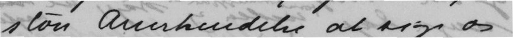stor Anerkendelse at sige os,
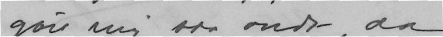gøre mig saa ondt, da
